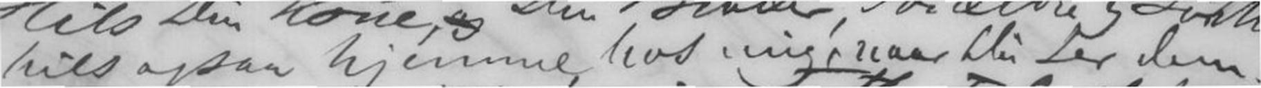hils ogsaa hjemme hos mig, naar Du ser dem.
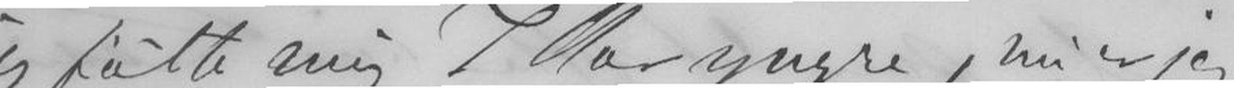jeg følte mig 7 Aar yngre, nu er jeg
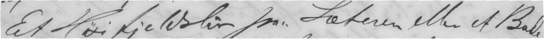Et Høifjeldsliv paa Sæteren eller et Bade
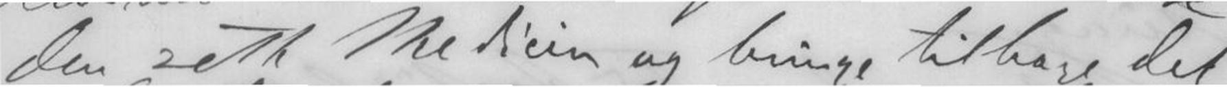den rette Medicin og bringe tilbage det
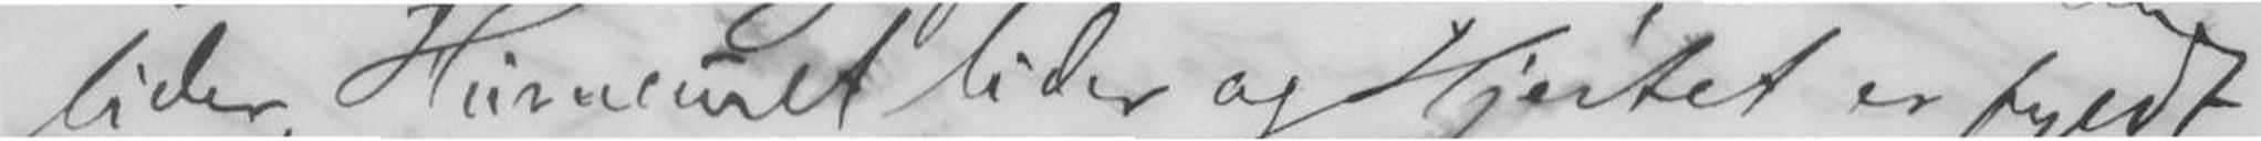lider, Humeuret lider og Hjertet er fyldt
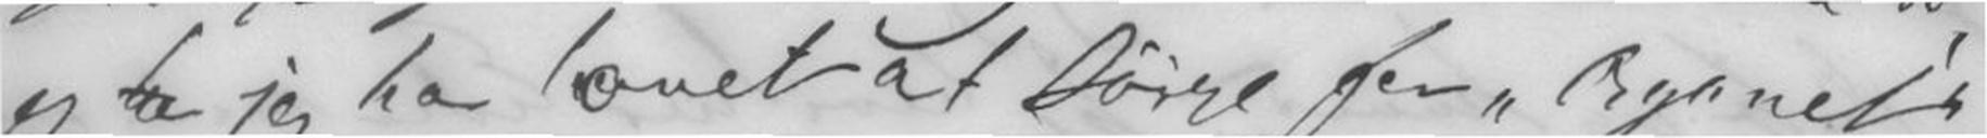og jeg har lovet at sørge for Organet
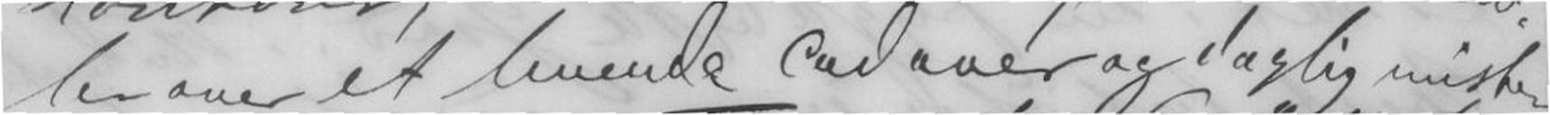ler over et levende Cadaver og daglig mister
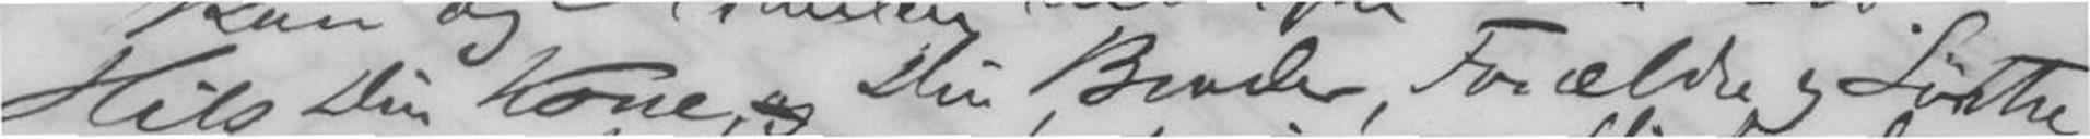Hils Din Kone, og Din Broder, Forældre og Søstre
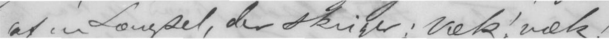af en Længsel, der skriger: væk! væk!
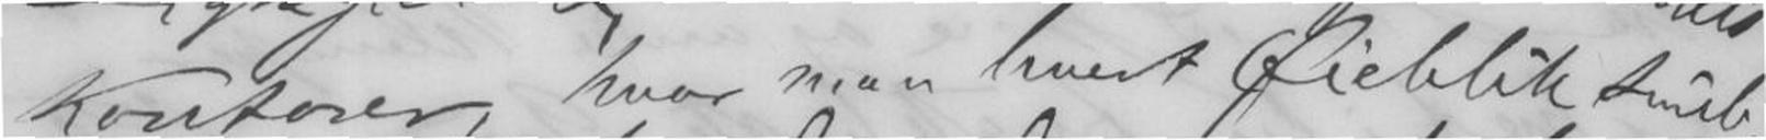Kontorer, hvor man hvert Øieblik snub
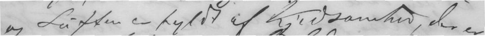og Luften er fyldt af Kjedsomhed, der er
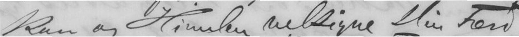kan og Himlen velsigne Din Færd.
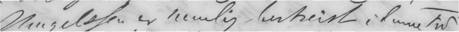Mengelssen er nemlig bortreist i denne Tid,
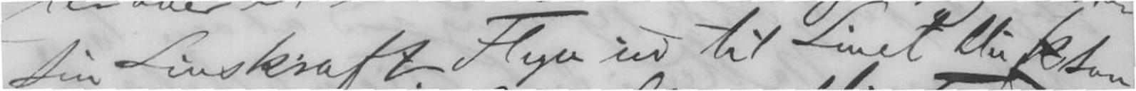sin Livskraft. Flyv ud til Livet Du k saa
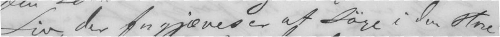Liv, der forgjæves er at søge i den store
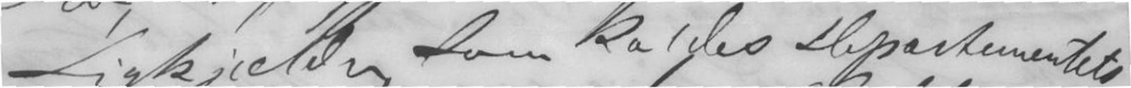Ligkjælder som kaldes Departementets
